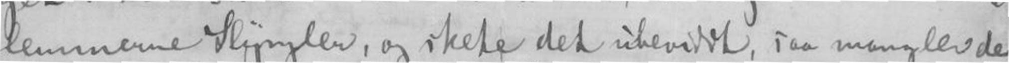lemmerne Slyngler, og skete det ubevidst, saa mangler de
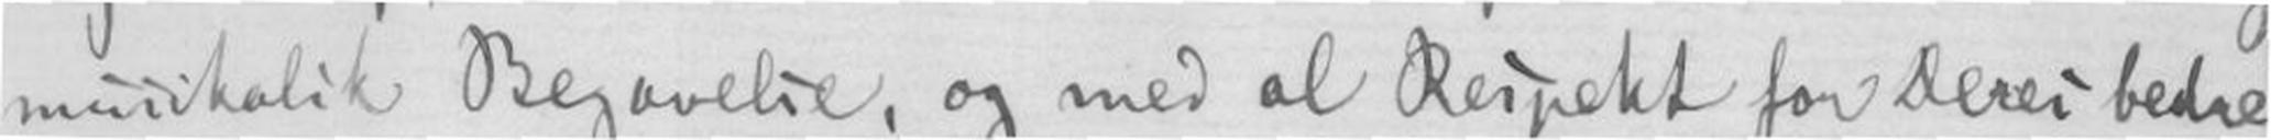musikalsk Begavelse, og med Respekt for Deres bedre
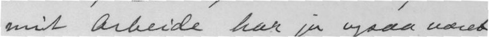mit Arbeide har jo ogsaa været
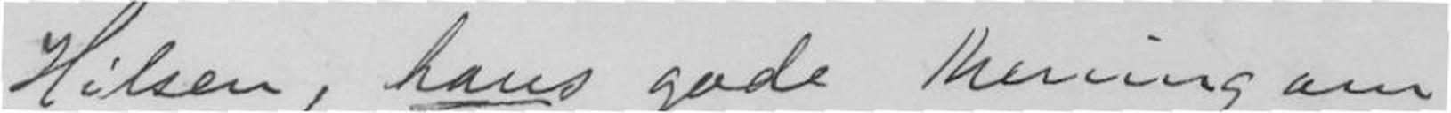Hilsen, hans gode Mening om
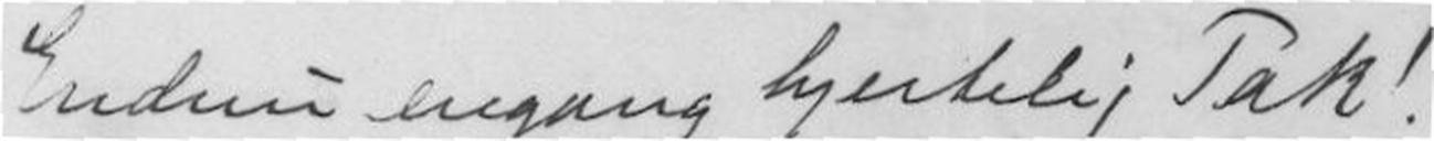Endnu engang hjertelig Tak!
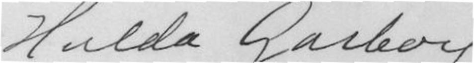Hulda Garborg
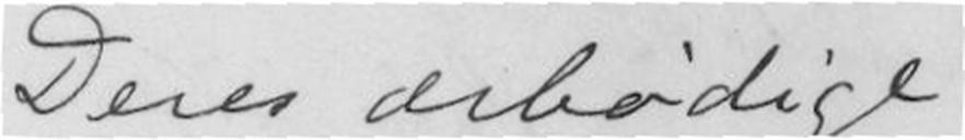Deres ærbødige
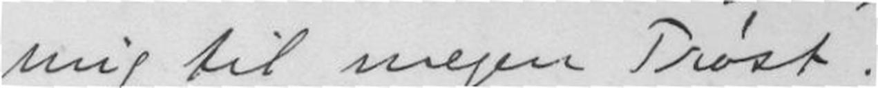mig til megen Trøst.
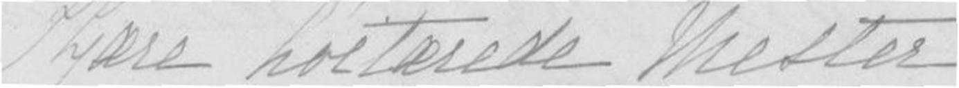Kjære høitærede Mester.
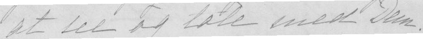at see og tale med Dem.
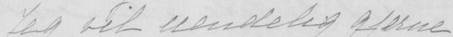Jeg vil uendelig gjerne
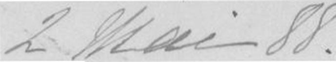2 Mai 88.
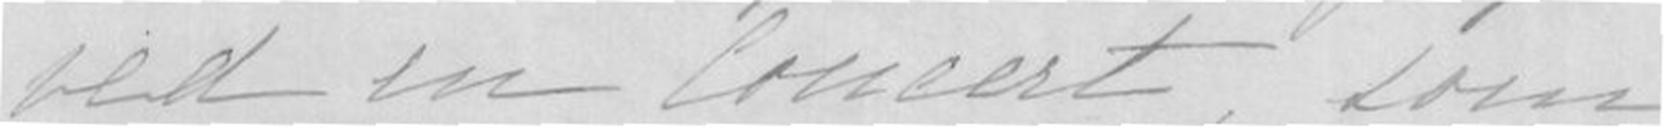ved en Concert, som
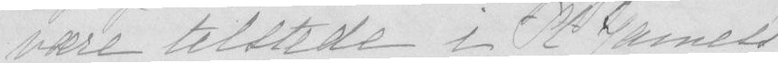være tilstede i St Jamess
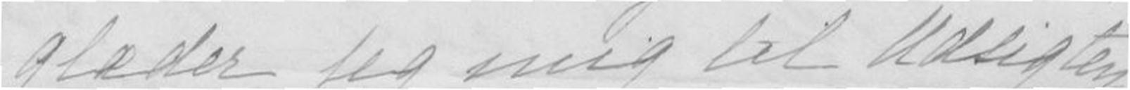glæder jeg mig til Udsigten
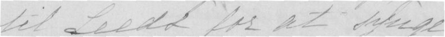til Leeds for at synge
